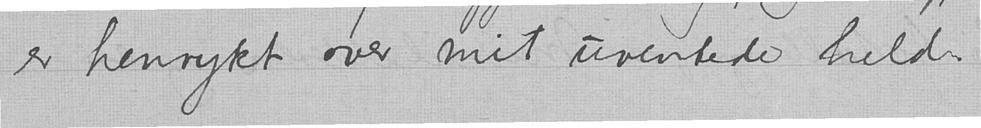er henrykt over mit uventede held.
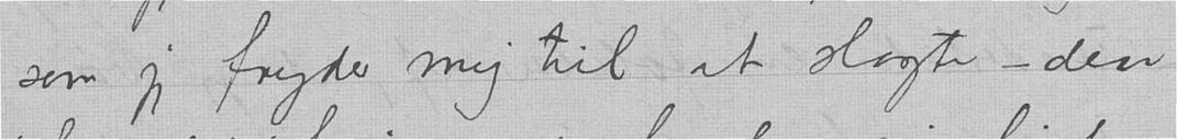som jeg fryder mig til at slagte — den
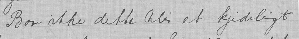Bare ikke dette blir et kjedeligt
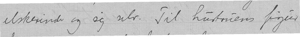elskerinde og sig selv. Til hustruens figur
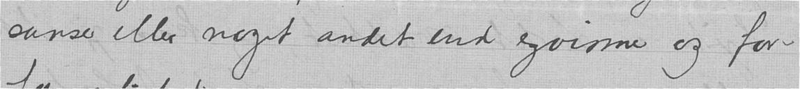sanser eller noget andet end egoisme og for-
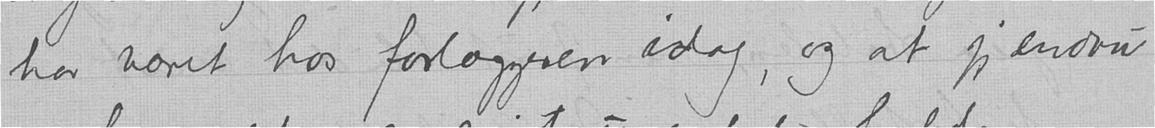har været hos forlæggeren idag, og at jeg endnu
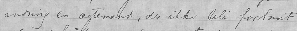andring en ægtemand, der ikke blir forstaat
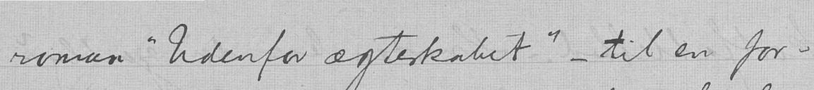roman "Udenfor ægteskabet" — til en for-
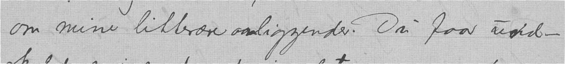om mine litterære anliggender. Du faar und-
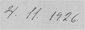4.11.1926.
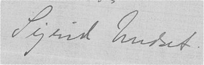Sigrid Undset.
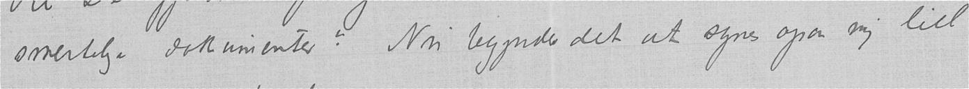smertelige dokumenter? Nu begynder det at synes ogsaa mig litt
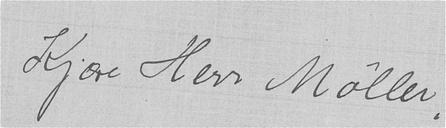Kjære Herr Møller,
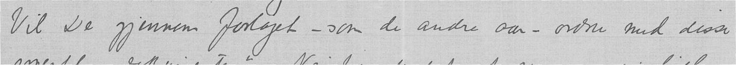Vil De gjennem forlaget – som de andre aar – ordne med disse
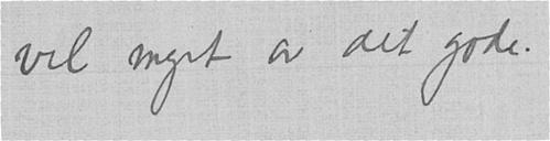vel meget av det gode.
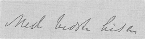Med bedste hilsen
